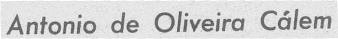Antonio de Oliveira Cálem
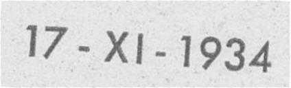17 -XI-1934
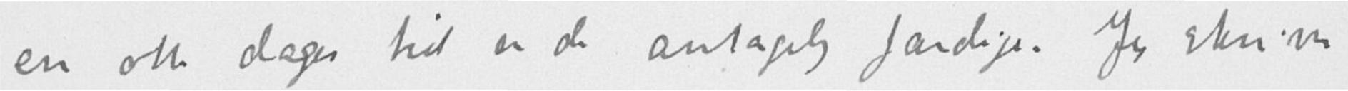en otte dagers tid er de antagelig færdige. Jeg skriver
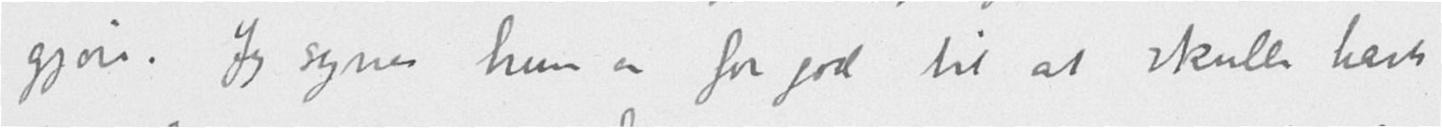gjøre. Jeg synes hun er for god til at skulle have
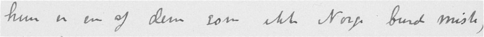hun er en af dem som ikke Norge burde miste,
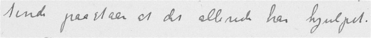tinde paastaar at det allerede har hjulpet.
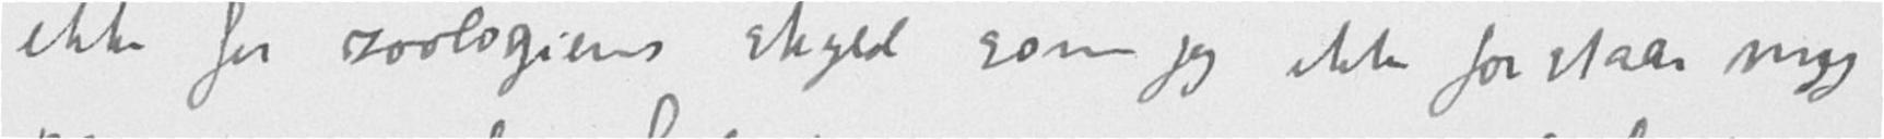ikke for zoologiens skyld som jeg ikke forstaar mig
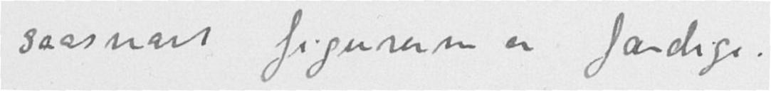saasnart figurerne er færdige.
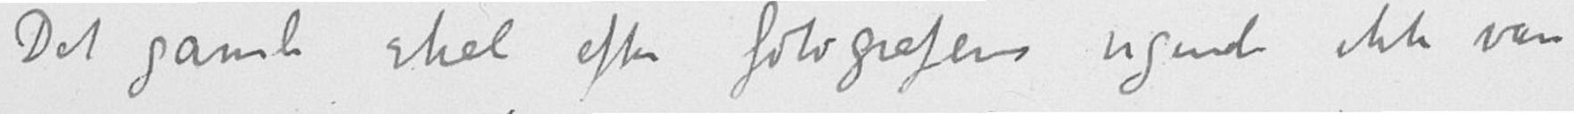Det gamle skal efter fotografens sigende ikke være
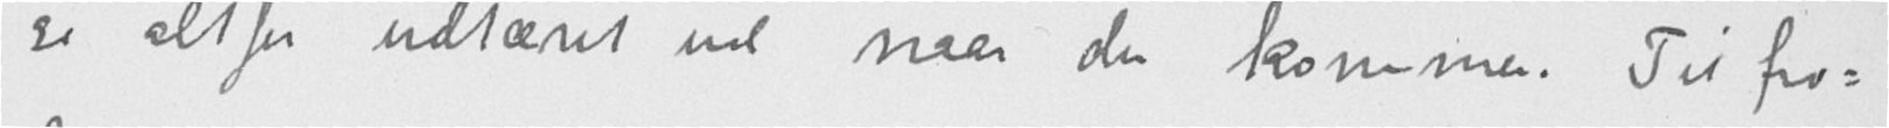se altfor udtæret ud naar du kommer. Til fro-
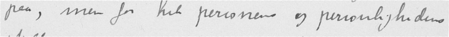paa, men for hele personens og personlighedens
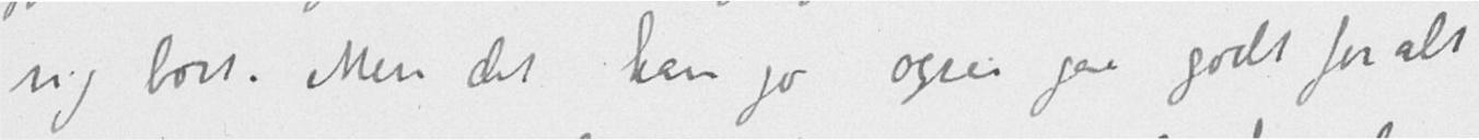sig bort. Men det kan jo ogsaa gaa godt for alt
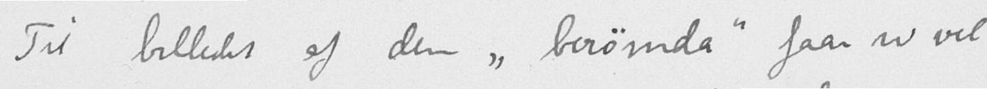Til billedet af den "berømda" faar vi vel
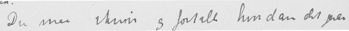Du maa skrive og fortælle hvordan det gaar
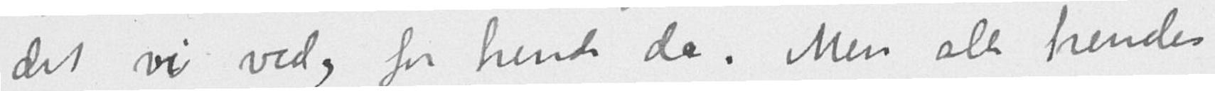det vi ved, for hende da. Men alle hendes
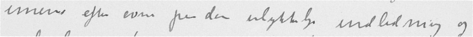imens efter som paa den ulykkelige indledning og
